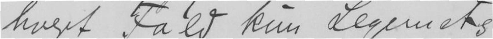hvert Fald kun Legemets
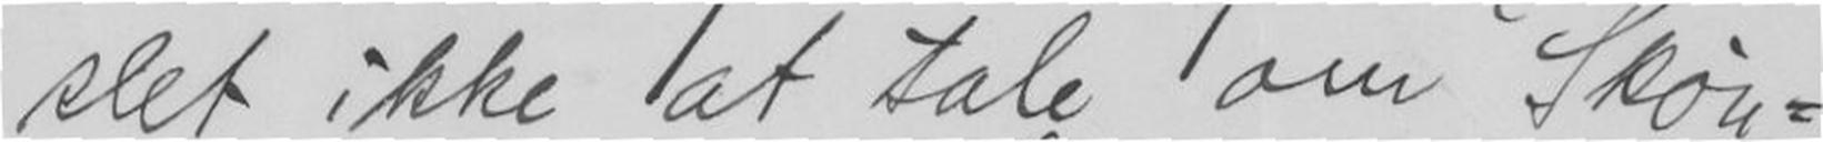slet ikke at tale om Skøn-
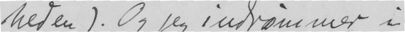heden). Og jeg indrømmer i
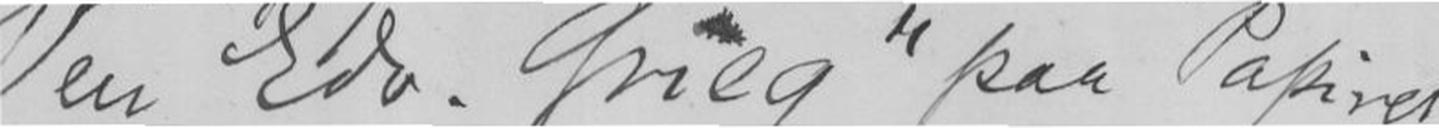Ven Edv. Grieg paa Papiret,
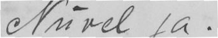Nuvel ja.
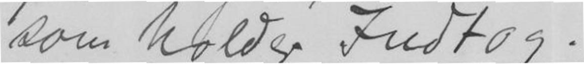som holder Indtog.
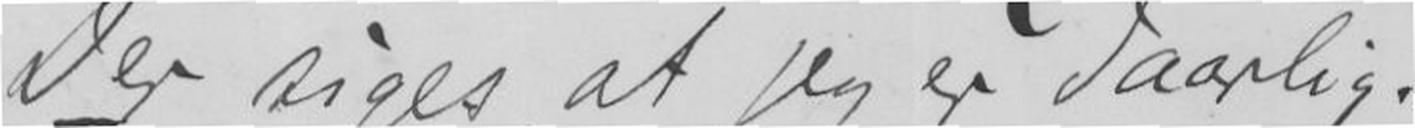Der siges, at jeg er daarlig.
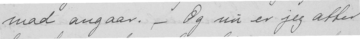mad angaar. - Og nu er jeg atter
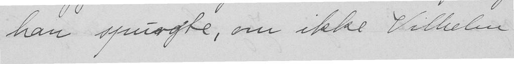han spurgte, om ikke Vilhelm
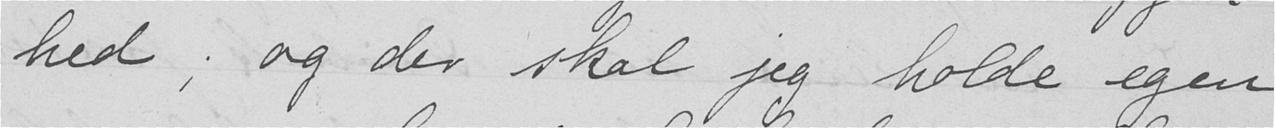hed; og der skal jeg holde egen
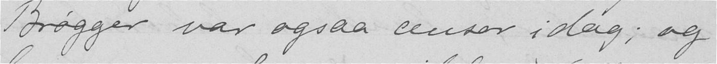Brøgger var ogsaa censor i dag; og
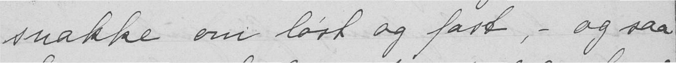snakke om løst og fast, - og saa
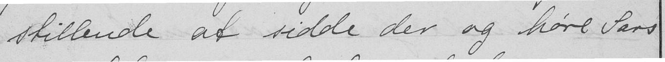stillende at sidde der og høre Sars
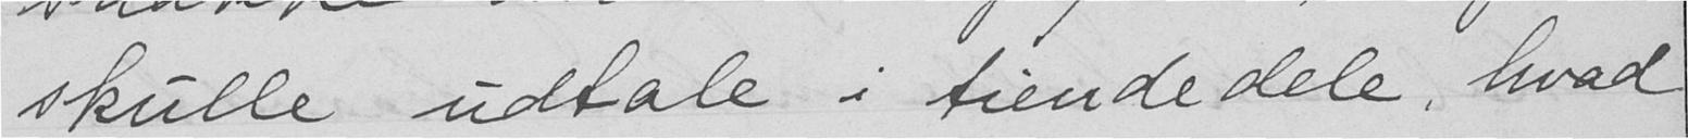skulle udtale i tiendedele, hvad
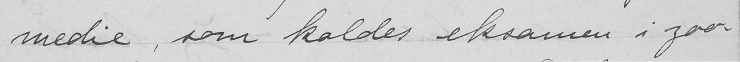medie, som kaldes eksamen i zoo-
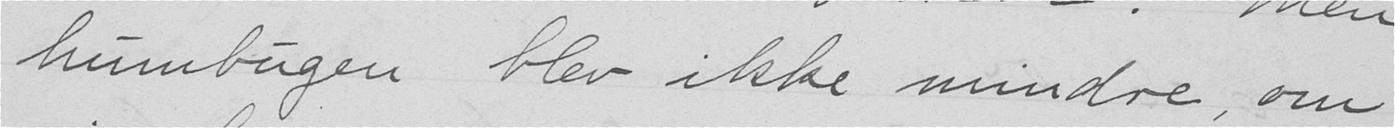humbugen blev ikke mindre, om
